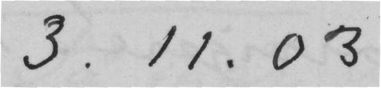3.11.03
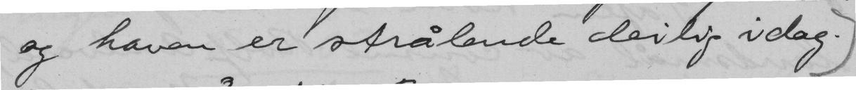og haven er strålende deilig idag.
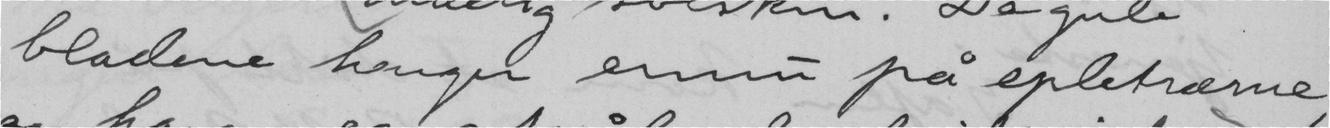bladene henger ennu på epletrærne,
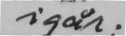i går.
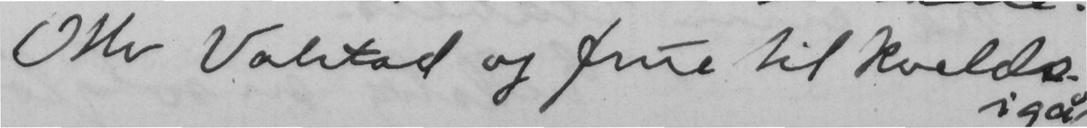Otto Vahtad og frue til kvelds
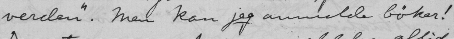verden". Men kan jeg anmelde bøker!
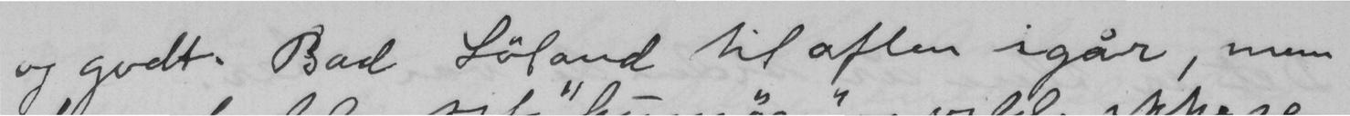og godt. Bad Løland til aften igår, men
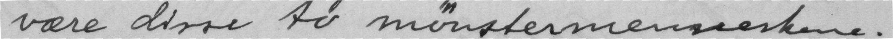være disse to mønstermenneskene.
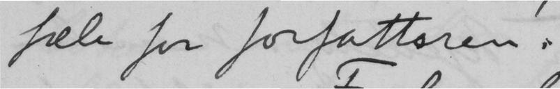fæle for forfatteren.
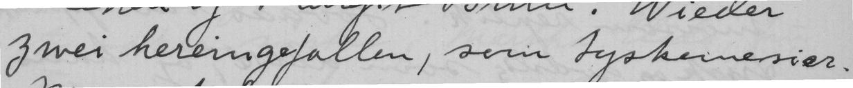zwei hereingefallen, som tyskerne sier.
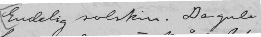Endelig solskin. De gule
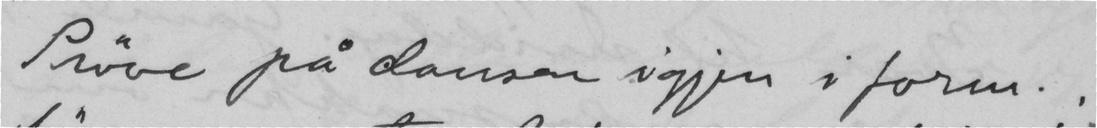Prøve på dansen igjen i form.;
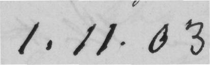1.11.03
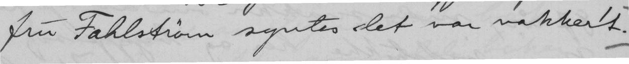fru Fahlstrøm syntes det var vakkert.
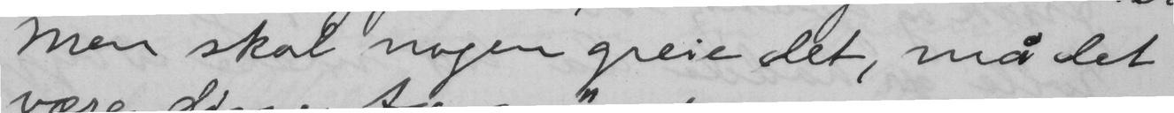Men skal nogen greie det, må det
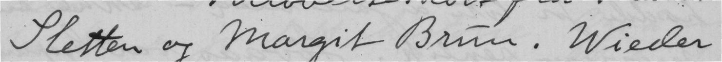Sletten og Margit Brun. Wieder
